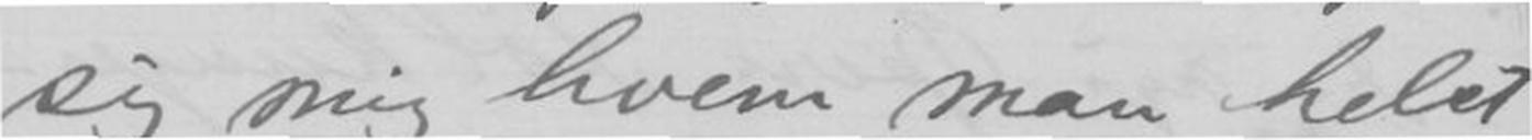sig mig hvem man helst
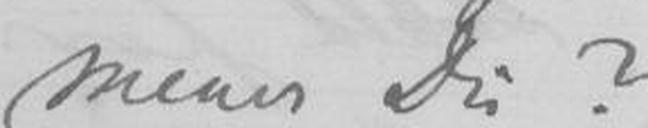mener Du?
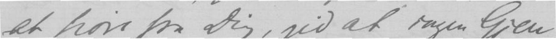at høre fra Dig, gid at inden Gjen-
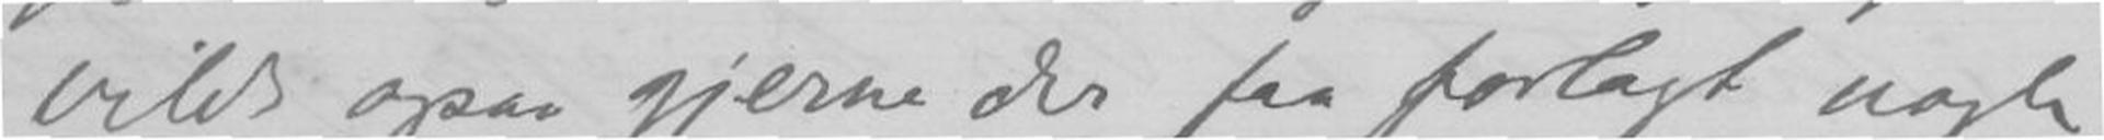vilde ogsaa gjerne der faa forlagt nogle
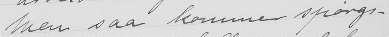Men saa kommer spørgs-
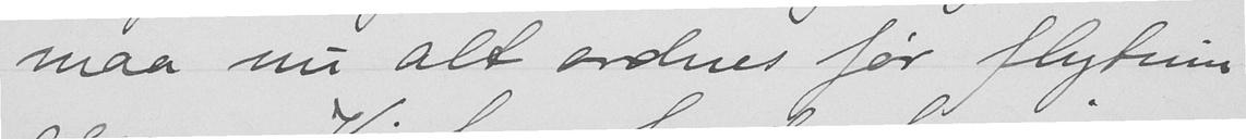maa nu alt ordnes før flytnin-
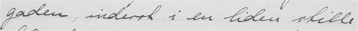gaden, inderst i en liden stille
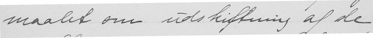maalet om udskiftning af de
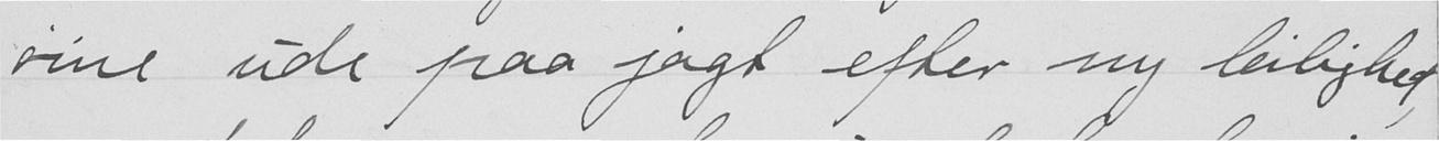øine ude paa jagt efter ny leilighed
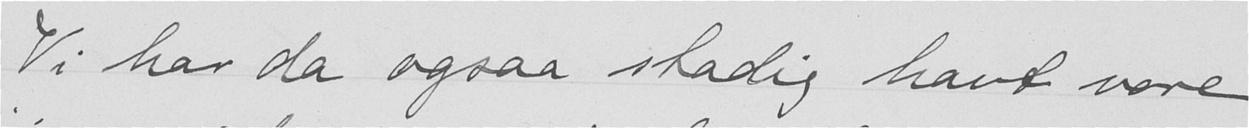Vi har da ogsaa stadig havt vore
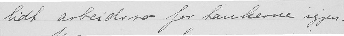lidt arbeidsro for tankerne igjen.
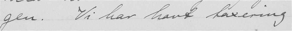gen. Vi har havt taxering
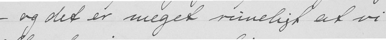- og det er meget rimeligt at vi
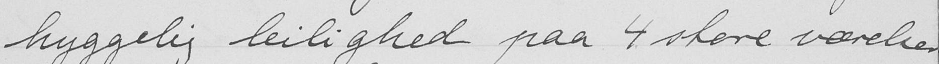hyggelig leilighed paa 4 store værelser
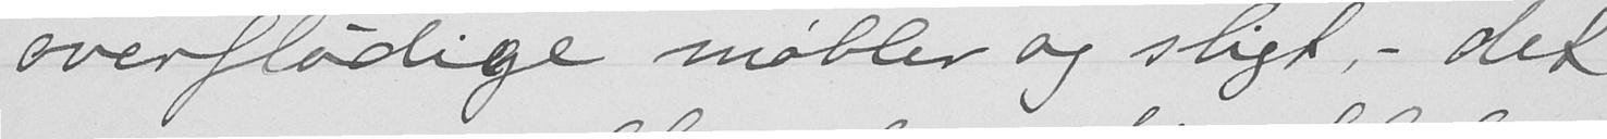overflødige møbler og sligt, - det
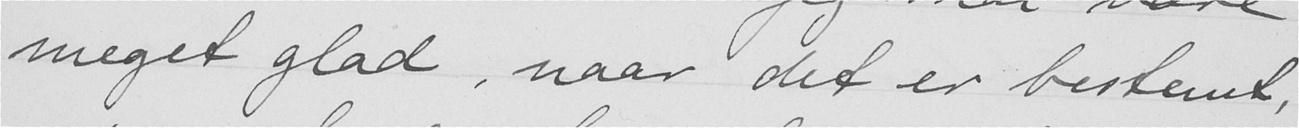meget glad, naar det er bestemt,
